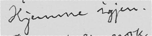Hjemme igjen.
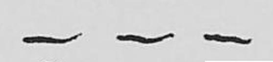- - -
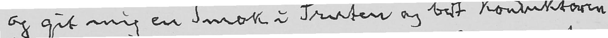og git mig en Smok i Truten og bedt Konduktøren
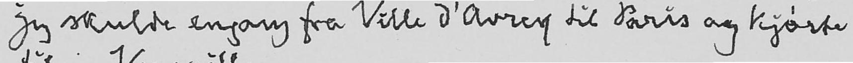jeg skulde engang fra Ville d'Avrey til Paris og kjørte
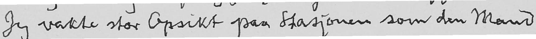Jeg vakte stor Opsikt paa Stasjonen som den Mand
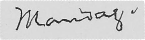Mandag.
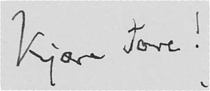Kjære Tore!
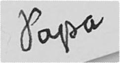Papa
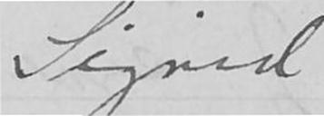Sigrid
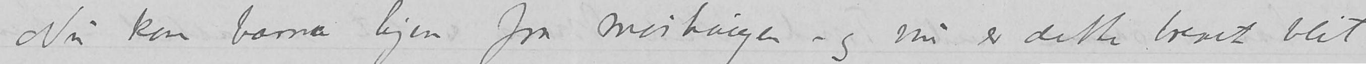Nu kom barna hjem fra maihaugen – og nu er dette brevet blit
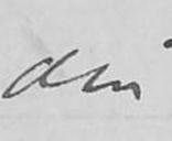din
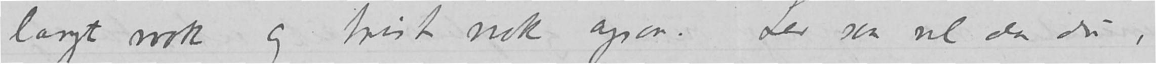langt nok og trist nok ogsaa.  Lev saa vel da du,
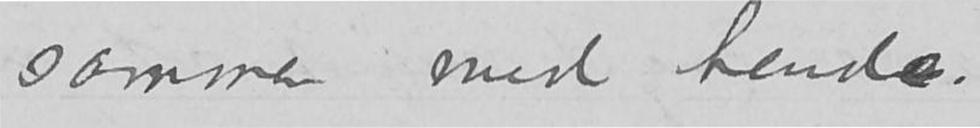sammen med hende.
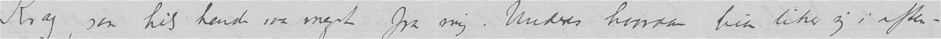Krag, saa hils hende saa meget fra mig.  Undres hvordan hun liker sig i aften-
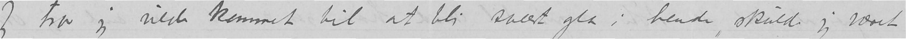Jeg tror jeg vilde kommet til at bli svært gla i hende, skulde jeg været
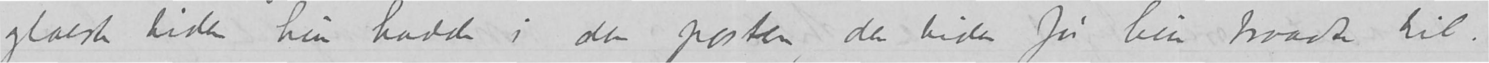glaeste tiden hun hadde i den posten, den tiden før hun traadte til.
